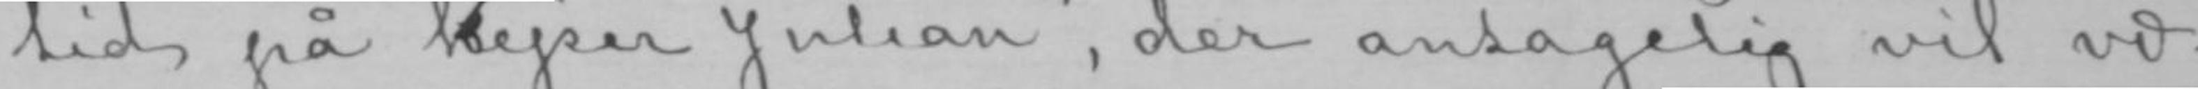tid på «kKejser Julian», der antagelig vil væ-
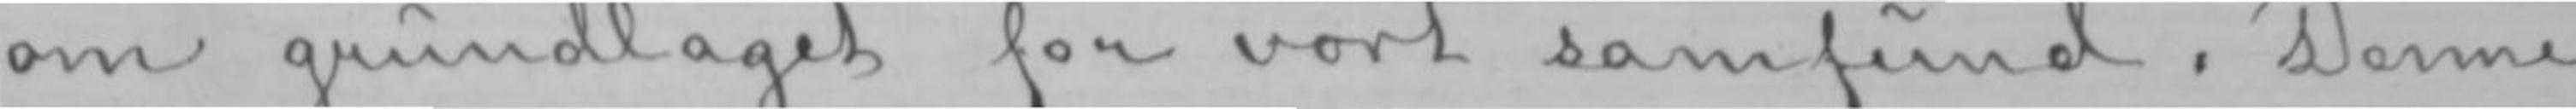om grundlaget for vort samfund. Denne
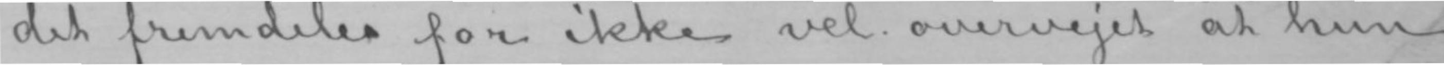det fremdeles for ikke vel overvejet at hun
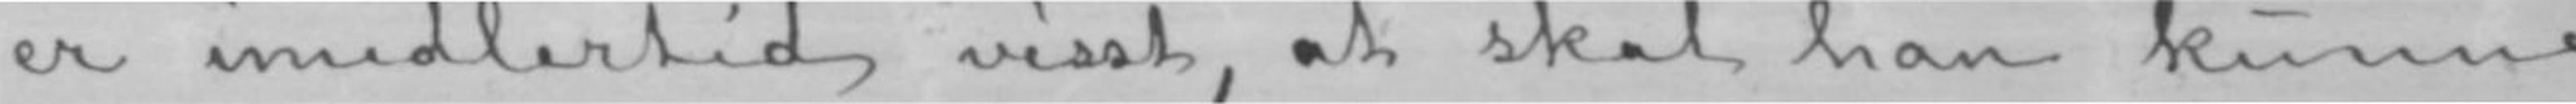er imidlertid visst, at skal han kunne
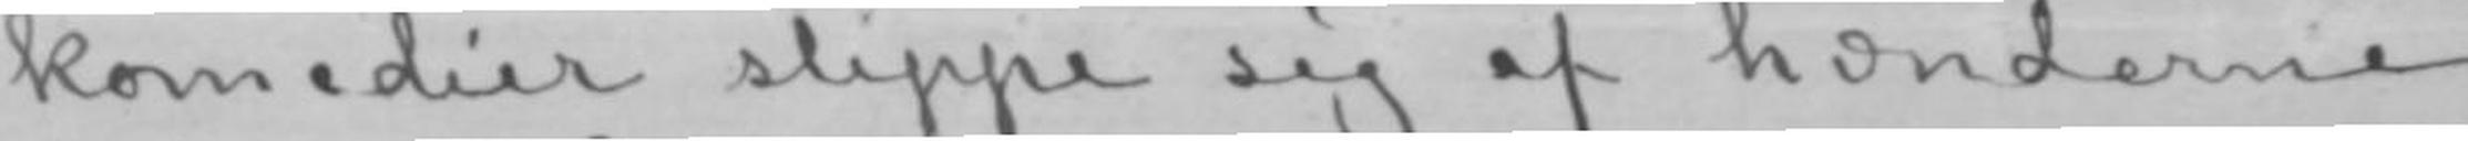komedier slippe sig af hænderne.-
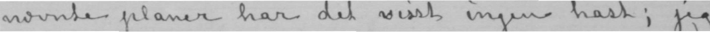nævnte planer har det visst ingen hast; jeg
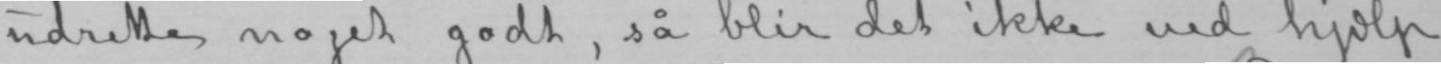udrette noget godt, så blir det ikke ved hjælp
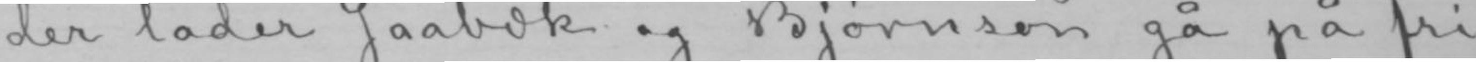der lader Jaabæk og Bjørnson gå på fri
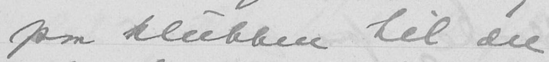paa klubben til ære
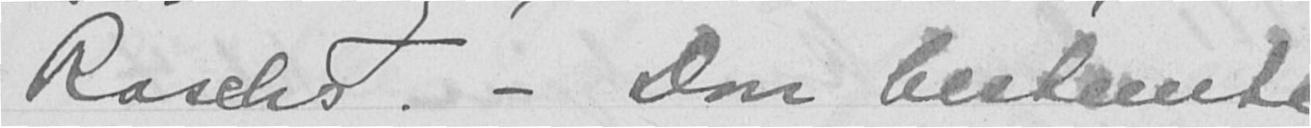Raschs. - Don bestemte
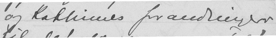og Kathrines forandringer
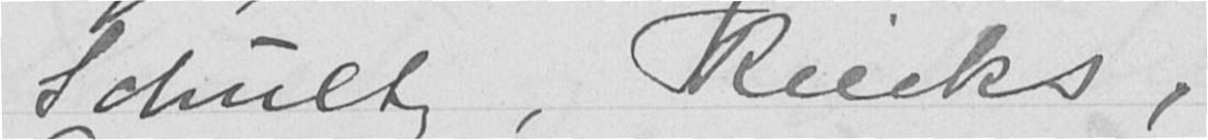Schultz, Riecks,
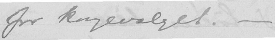for kongevalget. -
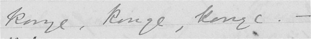konge, konge, konge. -
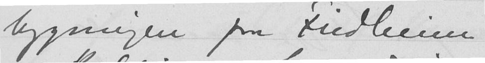bygningen paa Fridheim
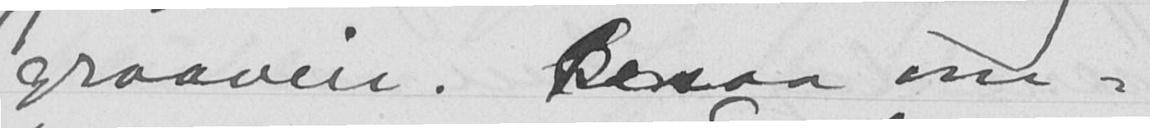graaveir. Besaa om-
